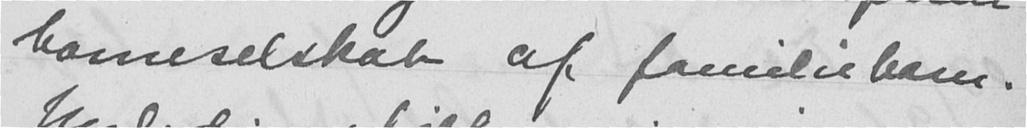barneselskab af familiebarn.
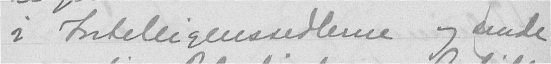i Intelligenssedlerne og sende
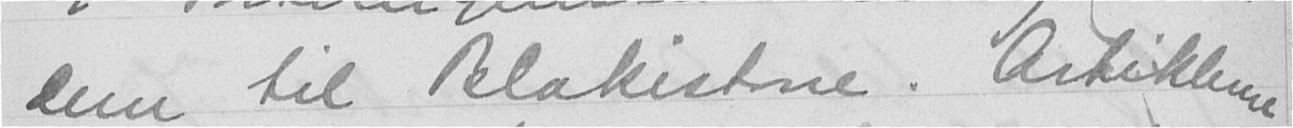dem til Blakistone. Artiklene
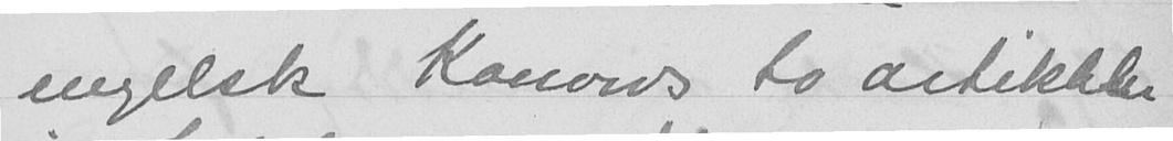engelsk Konows to artikkler
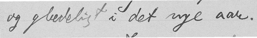og glædeligt i det nye aar.
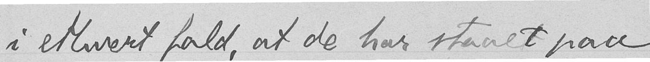i ethvert fald, at de har staaet paa
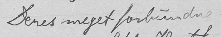Deres meget forbundne
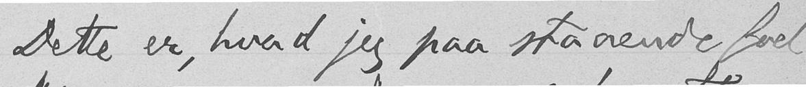Dette er, hvad jeg paa staaende fod
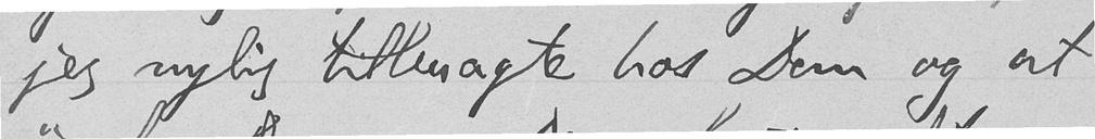jeg nylig tilbragte hos Dem og at
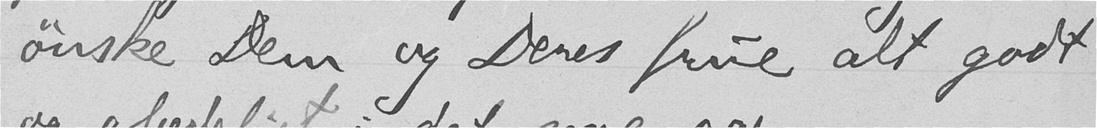ønske Dem og Deres frue alt godt
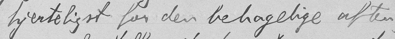hjerteligst for den behagelige aften,
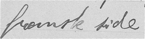fransk side.
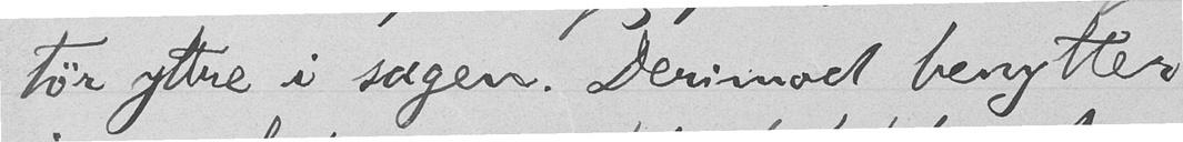tør yttre i sagen. Derimod benytter
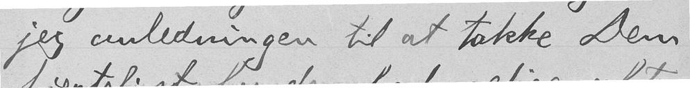jeg anledningen til at takke Dem
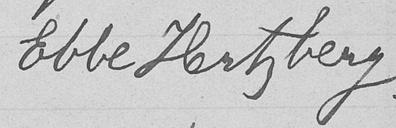Ebbe Hertzberg.
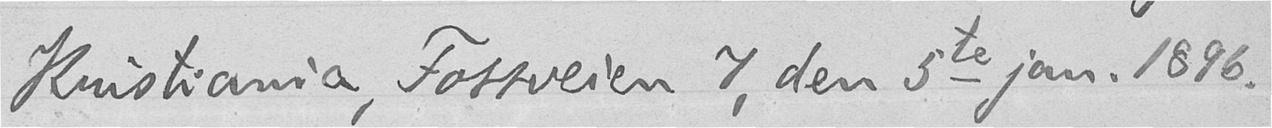Kristiania, Fossveien 7, den 5te jan. 1896.
